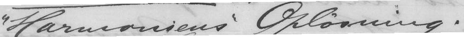"Harmoniens" Opløsning.
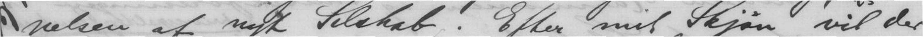nelsen af nyt Selskab. Efter mit Skjøn vil der
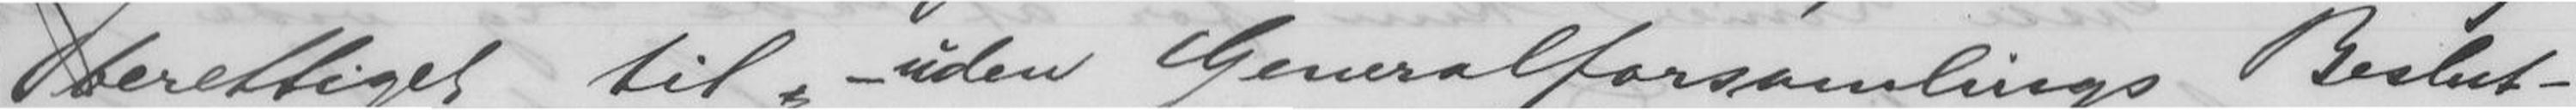berettiget til - uden Generalforsamlings Beslut-
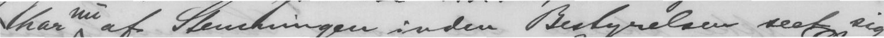har af Stemningen inden Bestyrelsen seet sig
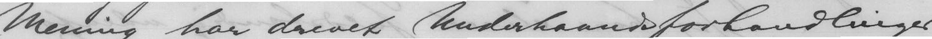Mening har drevet Underhaandsforhandlinger
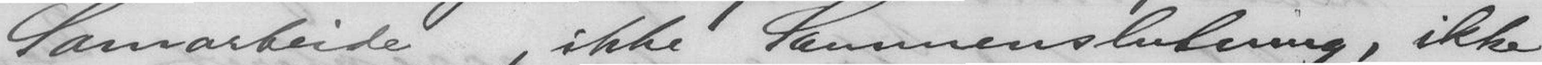Samarbeide, ikke Sammenslutning, ikke
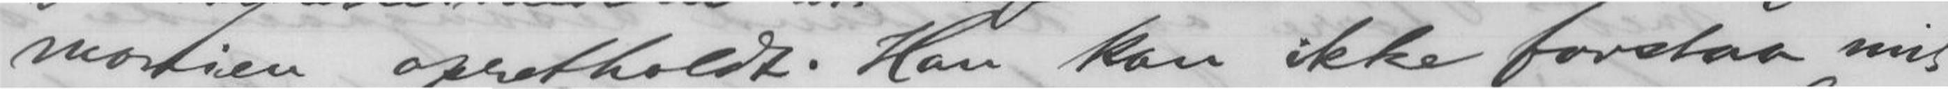monien opretholdt. Han kan ikke forstaa mit
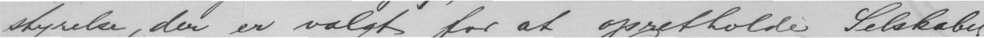styrelse, der er valgt for at opretholde Selskabet
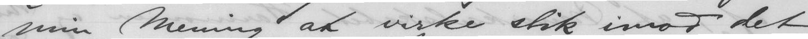min Mening at virke stik imod det,
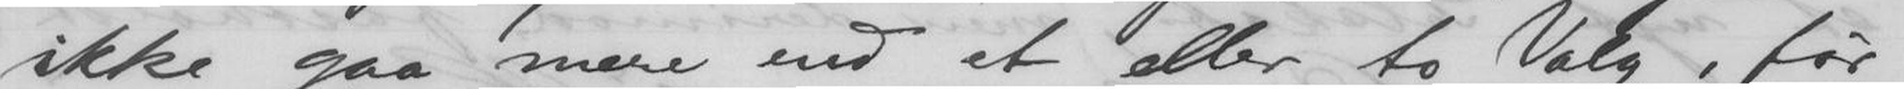ikke gaa mere end et eller to Valg, før
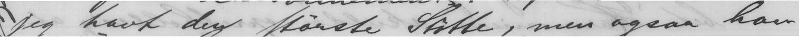jeg havt den største Støtte, men ogsaa han
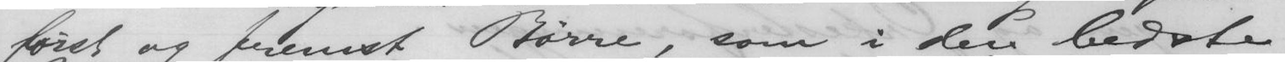først og fremst Børre, som i den bedste
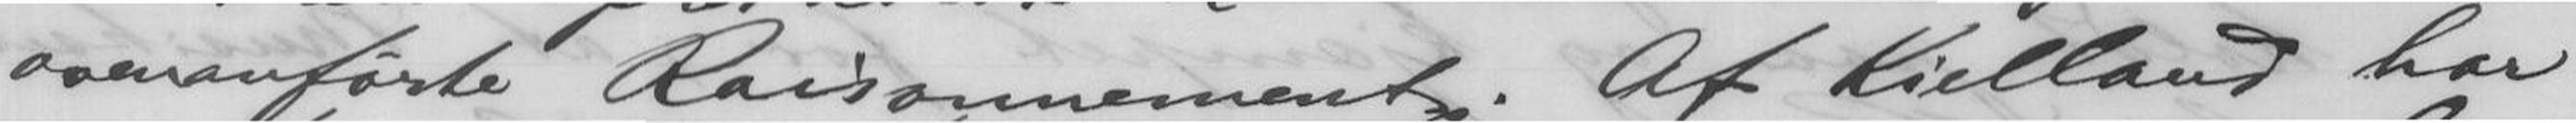ovenanførte Raisonnement. Af Kielland har
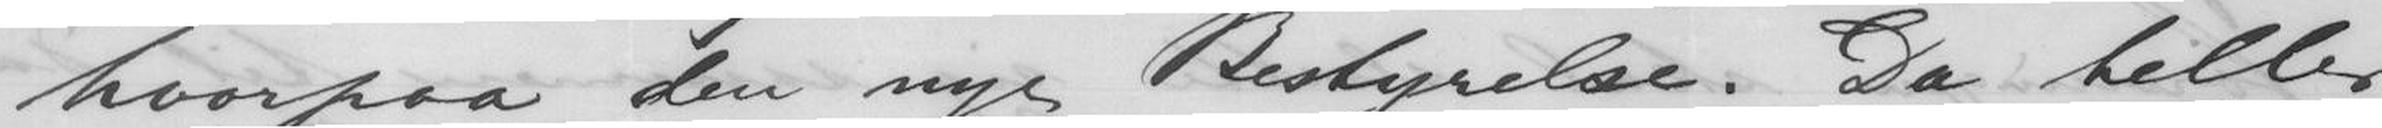hvorpaa den nye Bestyrelse. Da heller
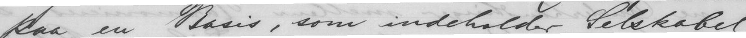paa en Basis, som indeholder Selskabet
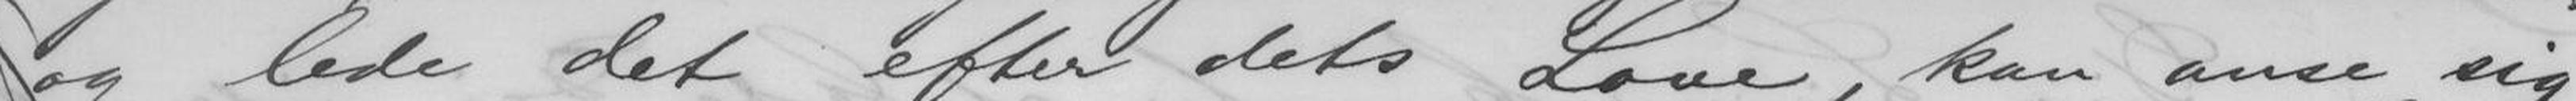og lede det efter dets Love, kan anse sig
